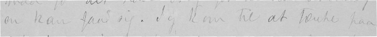en kan faa sig. Jeg kom til at tænke paa
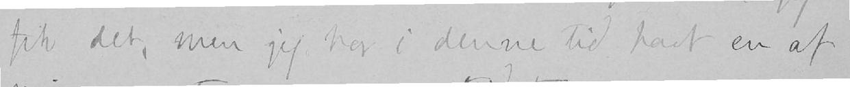fik det, men jeg har i denne tid havt en af
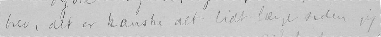brev, det er kanske alt lidt længe siden jeg
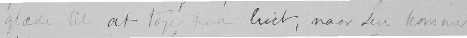glæde til at tage paa livet, naar Du kommer
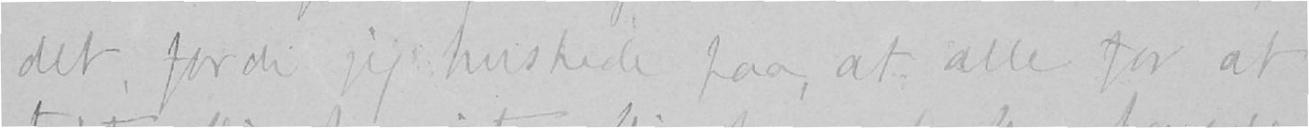det, fordi jeg huskede paa, at alle for at
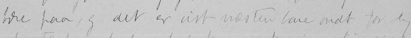bære paa, og det er vist næsten bare ondt for dig
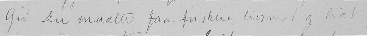Gid Du maatte faa friskere livsmod og lidt
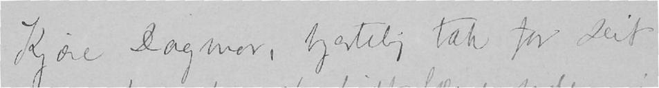Kjære Dagmar, hjertelig tak for Dit
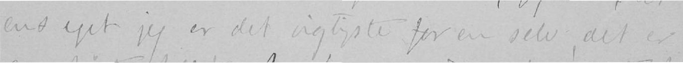ens eget jeg er det vigtigste for en selv, det er
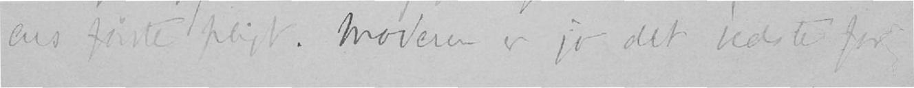ens første pligt. Moderen er jo det bedste for
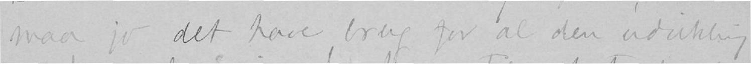maa jo det have brug for al den uvikling
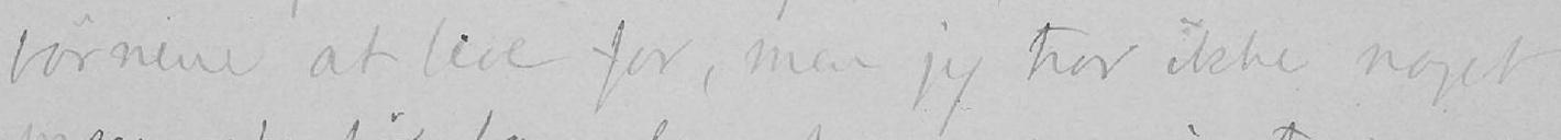børnene at leve for, men jeg tror ikke noget
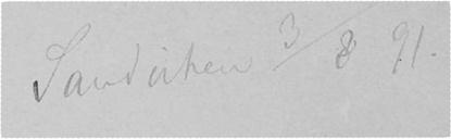Sandviken 3/8 91.
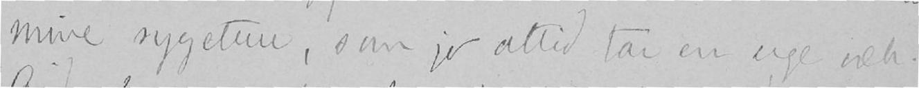mine sygeture, som jo altid tar en uge væk.
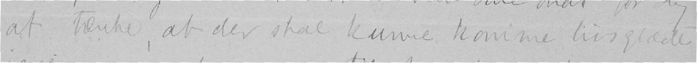at tænke, at der skal kunne komme livsglæde
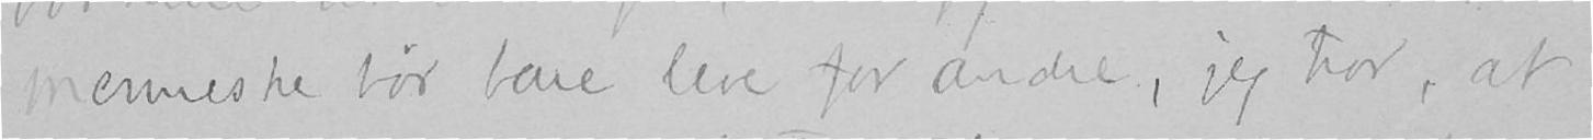menneske bør bare leve for andre, jeg tror, at
